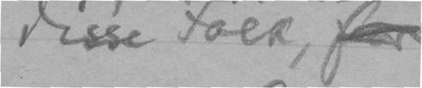disse Folk, for
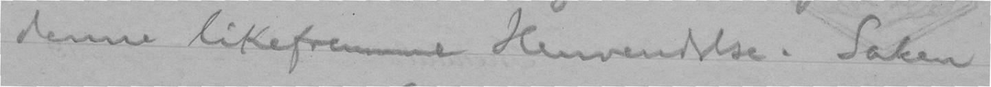denne likefremme Henvendelse. Saken
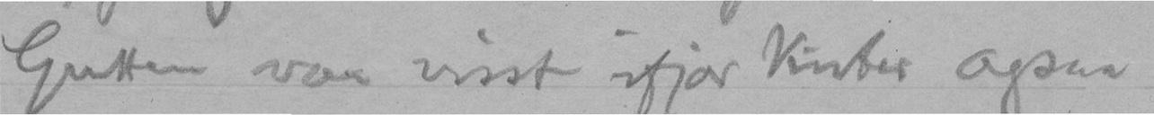Gutten var visst ifjor Vinter ogsaa
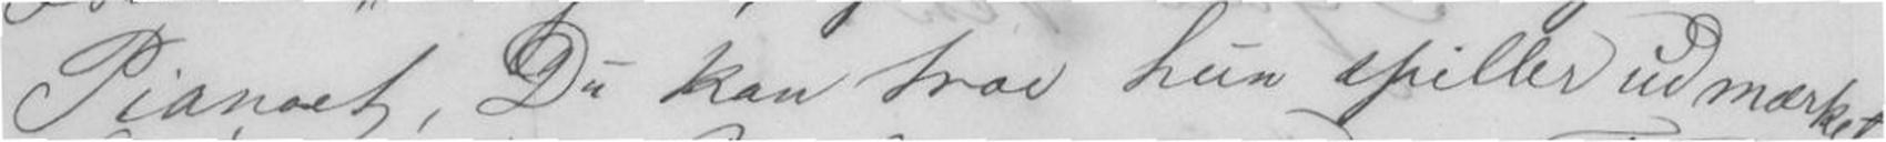Piano, Du kan troe hun spiller udmærket
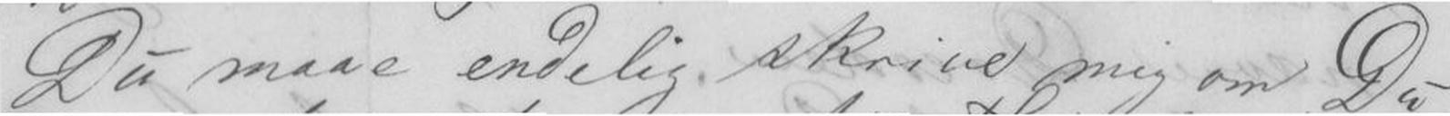Du maae endelig skrive mig om Du
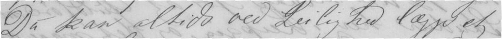Du kan altids ved Leilighed lægge et
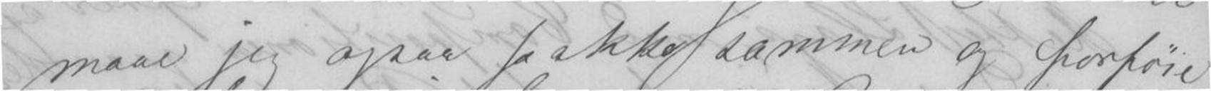maae jeg ogsaa pakke sammen og forføre
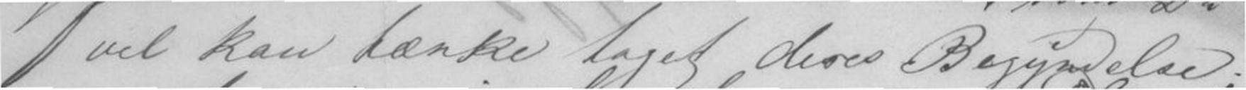vel kan tænke taget deres Begyndelse,
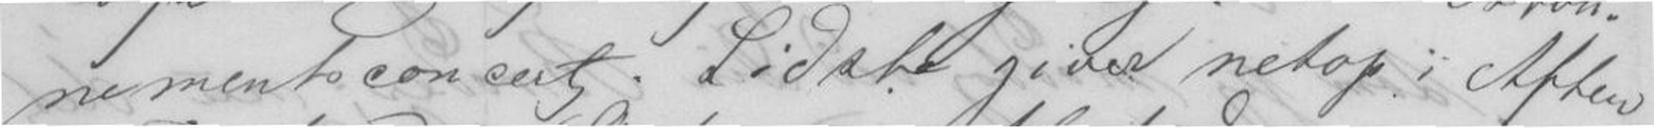nementsconcert. Sidste giver netop i Aften
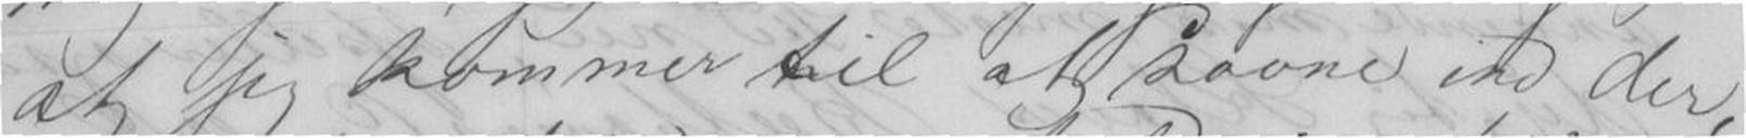at jeg kommer til at sovne ind der,
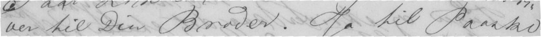ver til Din Broder. Ja til Paaske
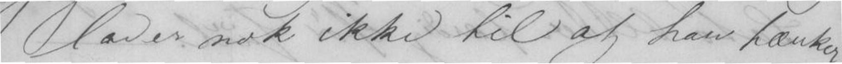lader nok ikke til at han tænker
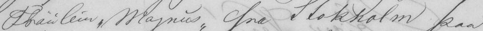Fräulein Magnus, fra Stokholm paa
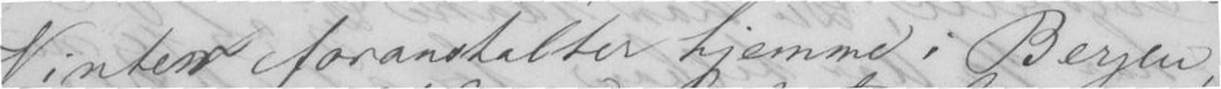Vinter foranstalter hjemme i Bergen,
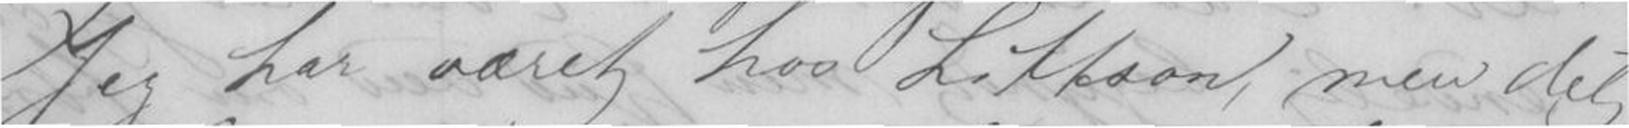Jeg har været hos Littson, men det
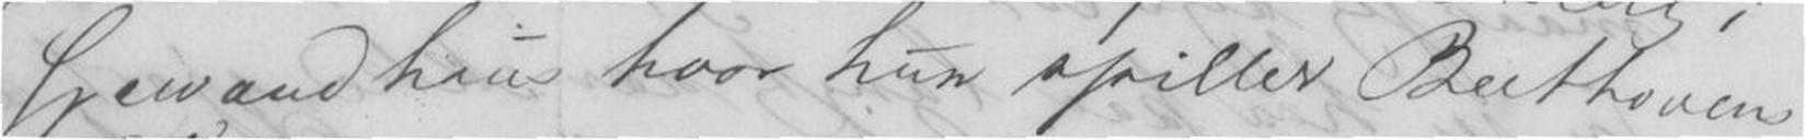Gewanthaus hvor hun spiller Beethoven
